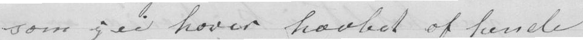som jei hover haabet of hende
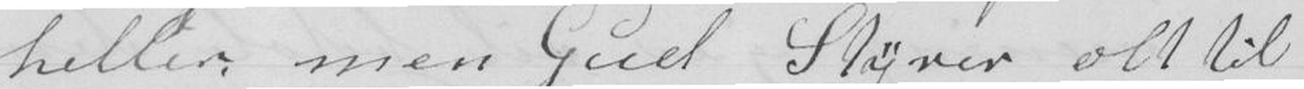heller. men Gud Styrer olt til
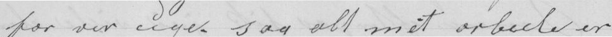for ver uge. saa alt mit orbede er
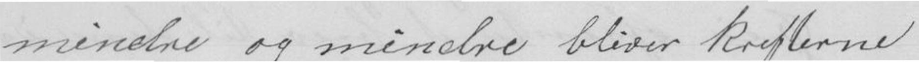mindre og mindre bliver krefterne
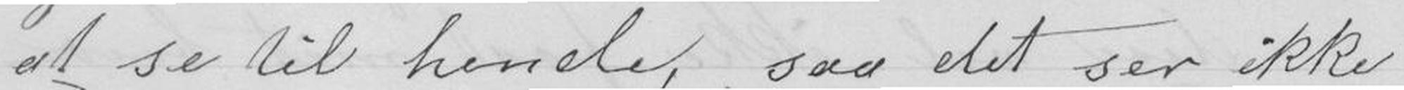at se til hende, saa det ser ikke
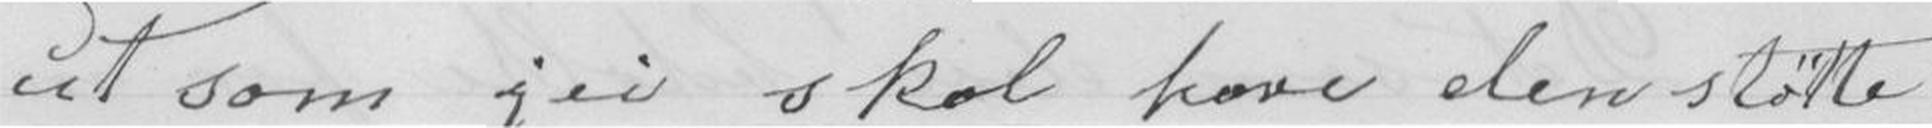ut som jei skal have den støtte
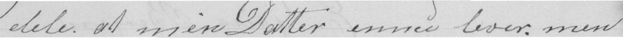dele at min Datter ennu lever. men
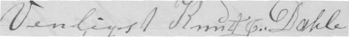Venligst Knut J. Dahle
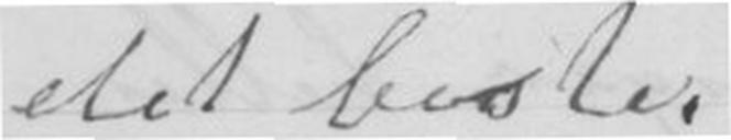det beste.
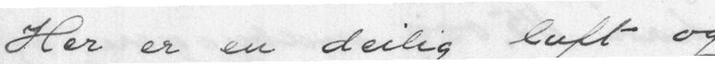Her er en deilig luft og
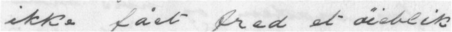ikke fået fred et øieblik
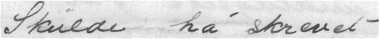Skulde ha' skrevet
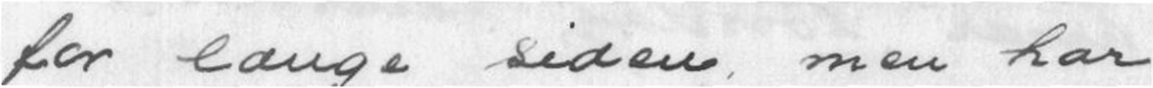for længe siden, men har
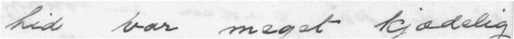hid var meget kjædelig
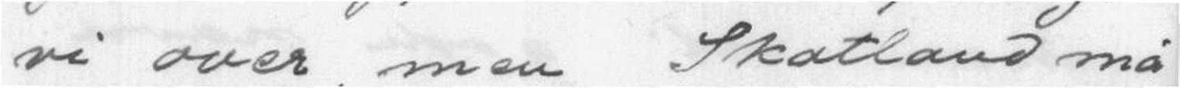vi over, men Skotland må
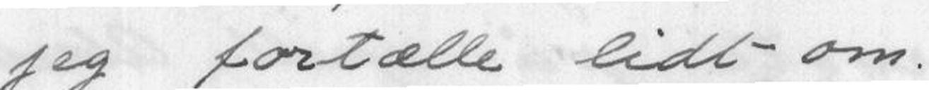jeg fortælle lidt om.
